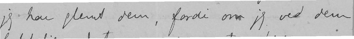jeg har glemt dem, fordi om jeg ved dem
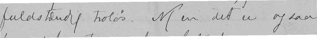fuldstændig troløs. Men det er ogsaa
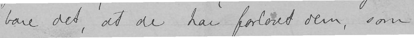bare det, at de har forlovet dem, som
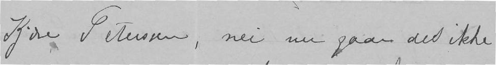Kjære Peterssen, nei nu gaar det ikke
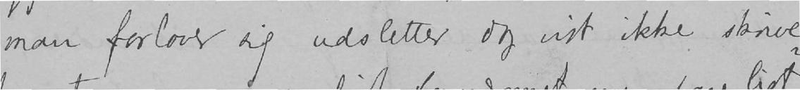man forlover sig udsletter dog vist ikke skrive-
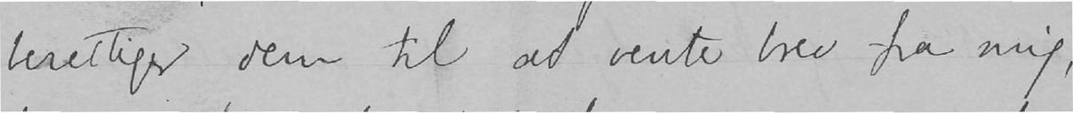berettiger dem til at vente brev fra mig,
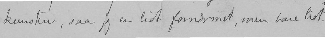kunsten, saa jeg er lidt fornærmet, men bare lidt.
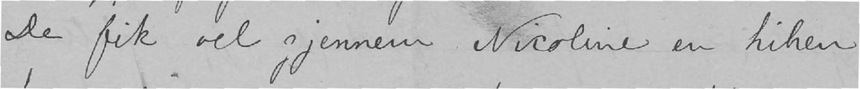De fik vel gjennem Nicoline en hilsen
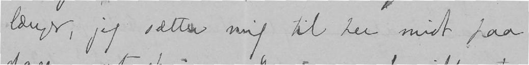længer, jeg sætter mig til her midt paa
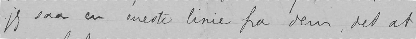jeg saa en eneste linie fra dem, det at
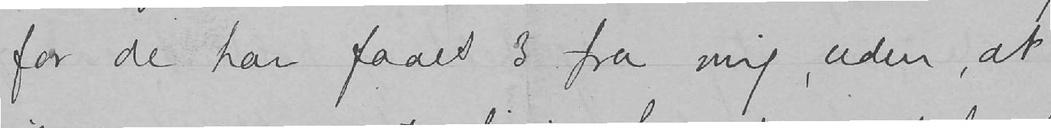for de har faaet 3 fra mig, uden, at
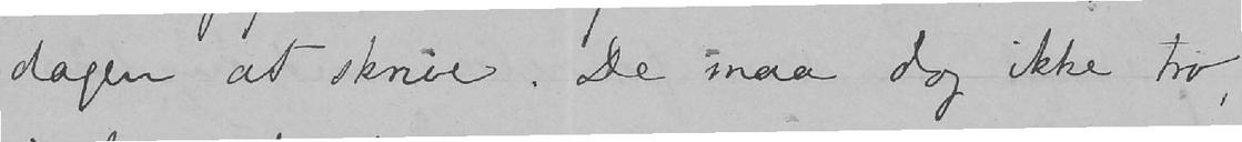dagen at skrive. De maa dog ikke tro,
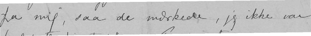fra mig, saa de mærkede, jeg ikke var
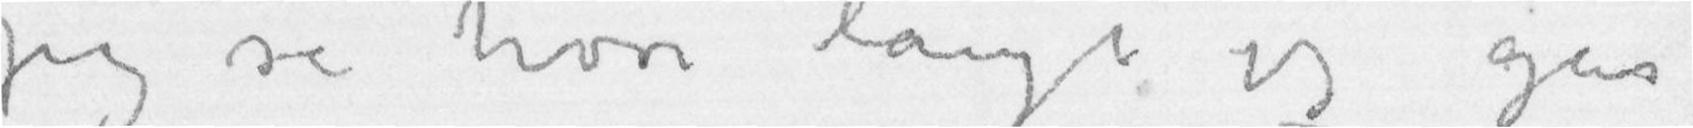jeg se hvor langt jeg går
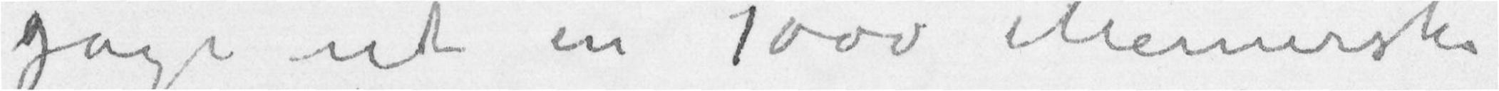jage ut en 1000 Mennesker
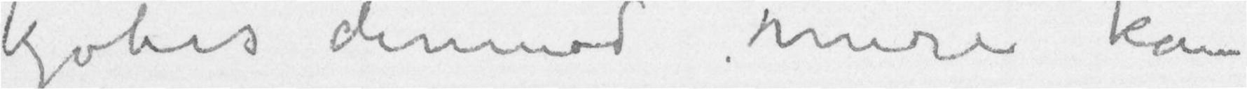Kjøbes derimod mere kan
